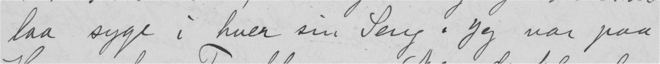laa syge i hver sin Seng. Jeg var paa
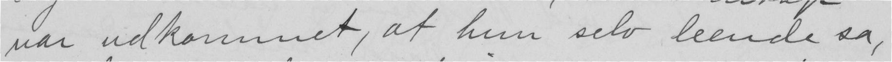var udkommet, at hun selv leende sa,
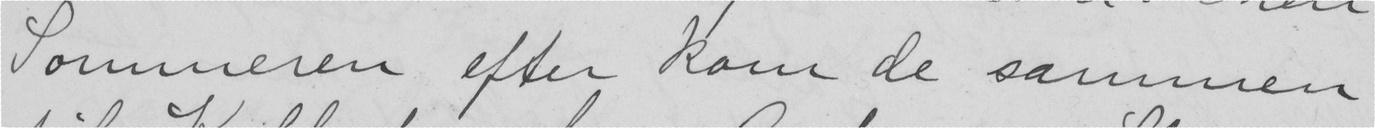Sommeren efter kom de sammen
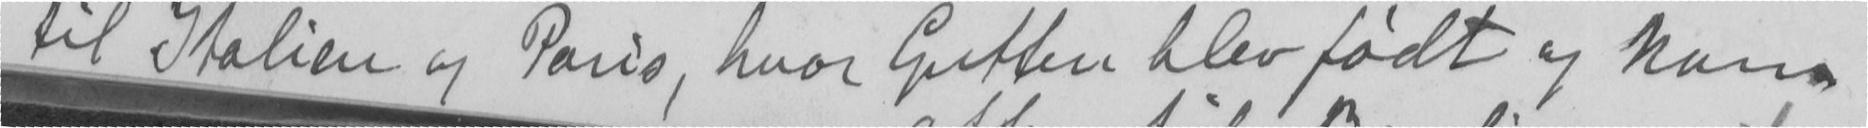til Italien og Paris, hvor Gutten blev født og kom
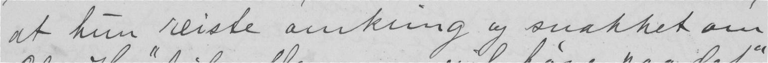at hun reiste omkring og snakket om
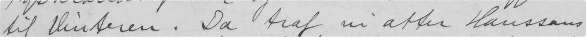til Vinteren. Da traf vi atter Hanssons
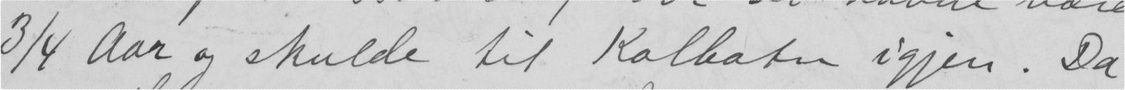3/4 Aar og skulde til Kolbotn igjen. Da
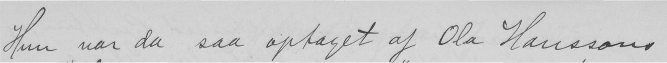Hun var da saa optaget af Ola Hanssons
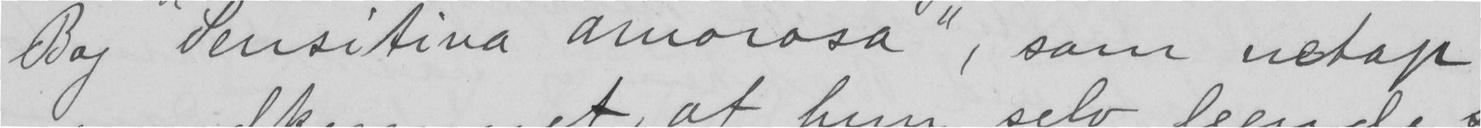Bog "Sensitiva amorosa", som netop
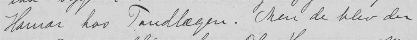Hamar hos Tandlægen. Men de blev der
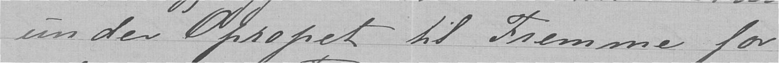under Opropet til Fremme for
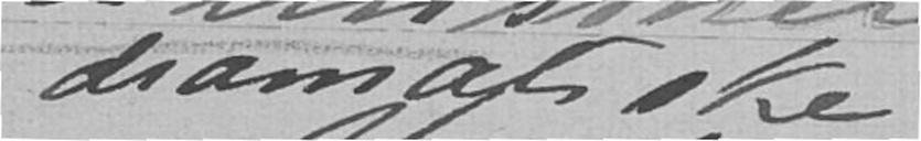dramatiske
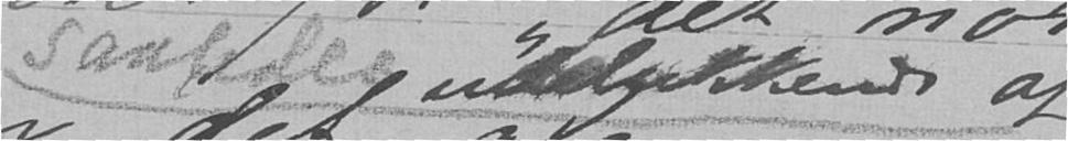sandelig udelukkende af
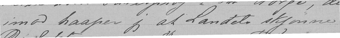imod haaper jeg at Landele skjønne
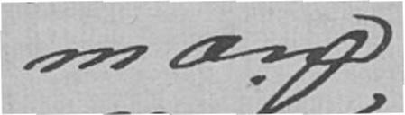mænd
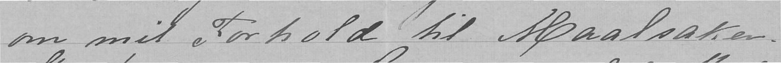om mit Forhold til Maalsaken.
